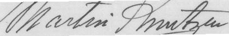Martin Knutzen
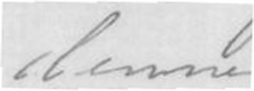denne
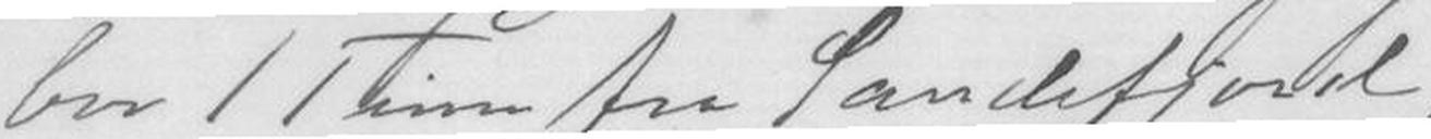bor 1 Time fra Sandefjord,
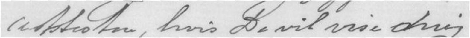Attesten, hvis De vil vise Mig
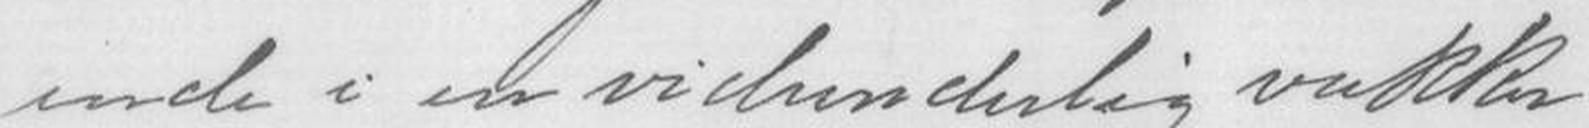inde i en vidunderlig vakker
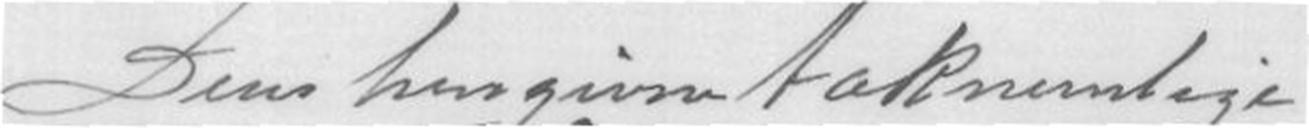Deres hengivne taknemlige
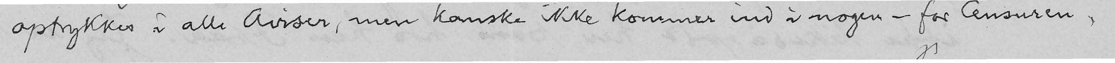optrykkes i alle Aviser, men kanske ikke kommer ind i nogen - for Censuren.
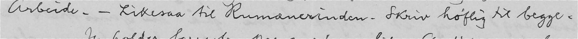Arbeide. - Likesaa til Rumænerinden. Skriv høflig til begge.
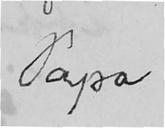Papa
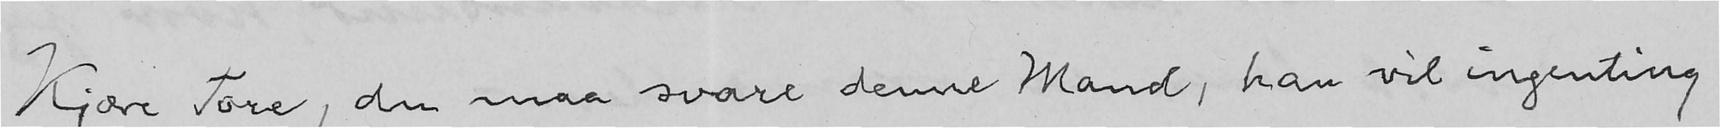Kjære Tore, du maa svare denne Mand, han vil ingenting
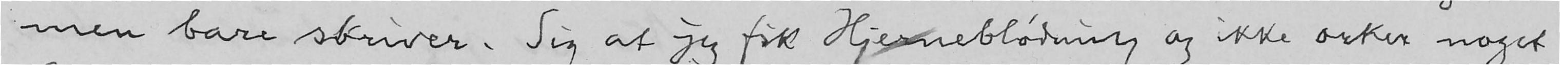men bare skriver. Sig at jeg fik Hjerneblødning og ikke orker noget
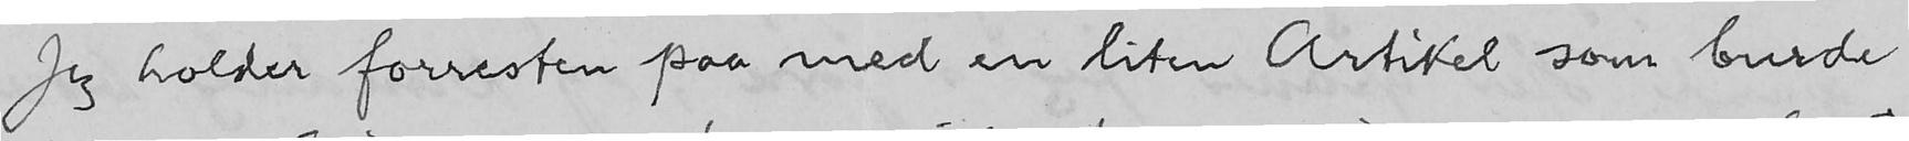Jeg holder forresten paa med en liten Artikel som burde
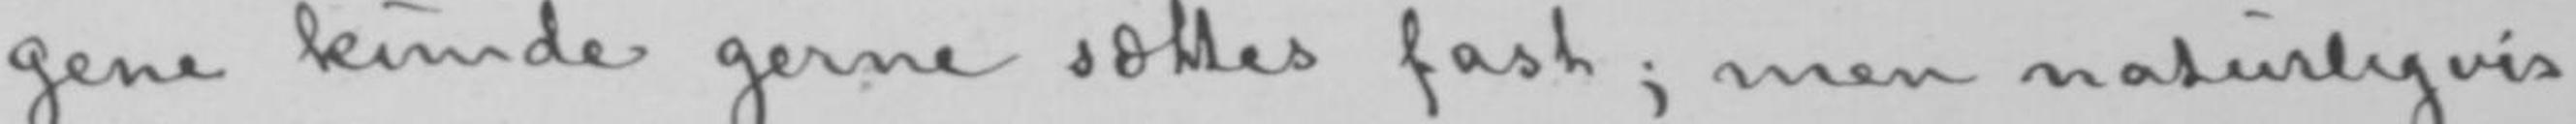gene kunde gerne sættes fast; men naturligvis
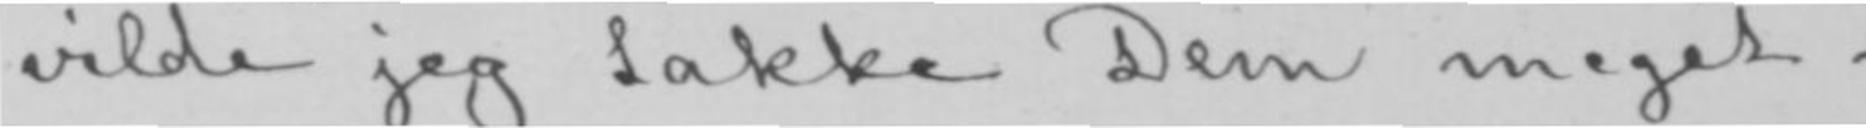vilde jeg takke Dem meget.
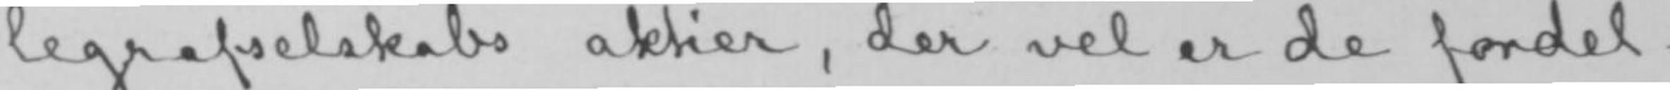legrafselskabs aktier, der vel er de fordel-
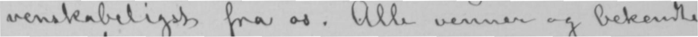venskabeligst fra os. Alle venner og bekendte
